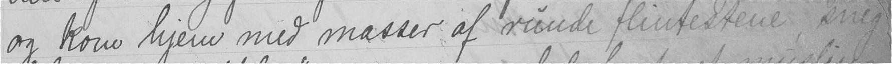og kom hjem med masser af runde flintestene, sneg-
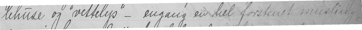lehuse og "vettelys" - engang en hel forstenet musling,
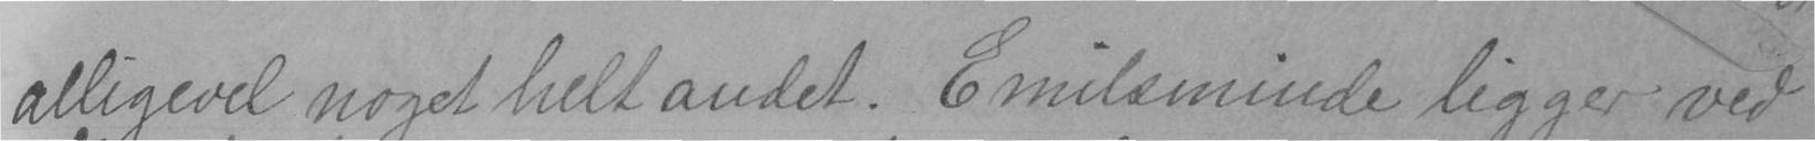alligevel noget helt andet. Emilsminde ligger ved
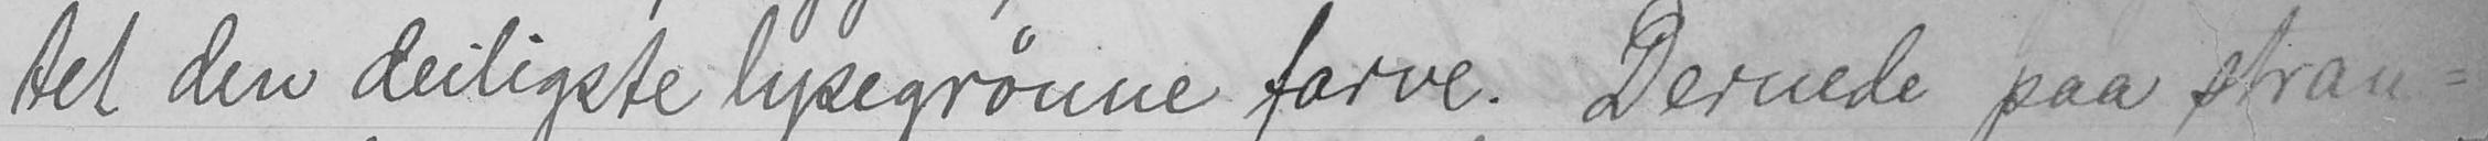seg den deiligste lypsegrønne farve. Dernede paa stran-
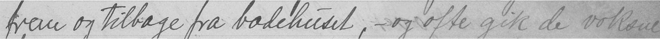frem og tilbage fra badehuset, - og ofte gik de voksne
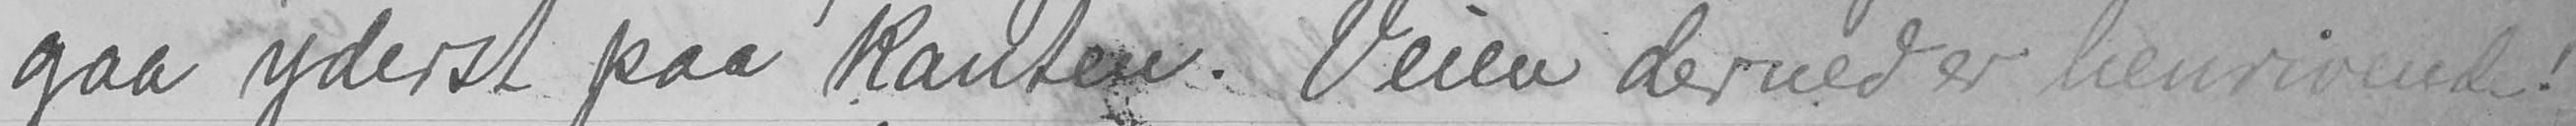gaa yderst paa kanten. Veien derned er henrivende!
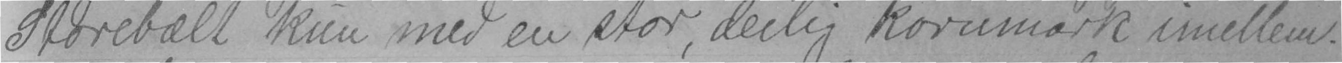Storebælt kun med en stor, deilig kornmark imellem.
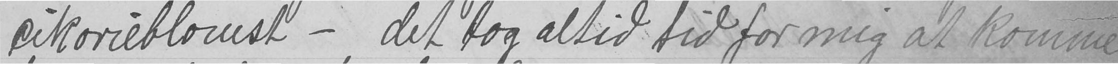cikorieblomst - det tog altid tid for mig at komme
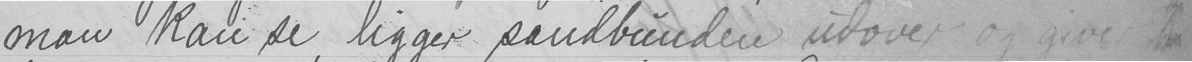man kan se, ligger sandbunden udover og giver fra
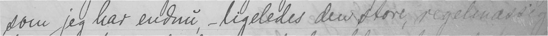som jeg har endnu, - ligeledes den store, regelmæssig
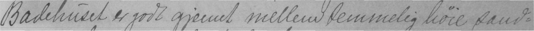Badehuset er godt gjemet mellem temmelig høie sand-
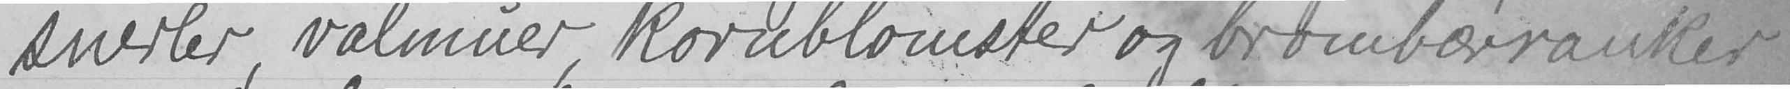snerler, valmuer, kornblomster og brombærranker
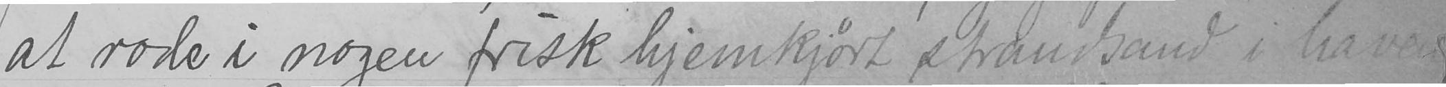at rode i nogen frisk hjemkjørt strandsand i haven
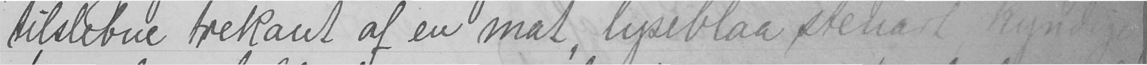tilstebne trekant af en mat, lyseblaa stenart kyndige
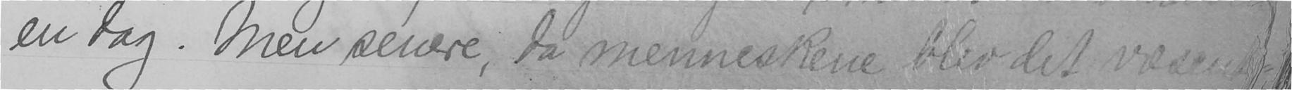en dag. Men senere, da menneskene blev det væsent-
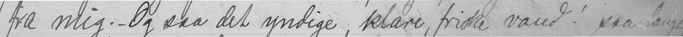fra mig. - Og saa det yndige, klare, friske vand! saa langt,
